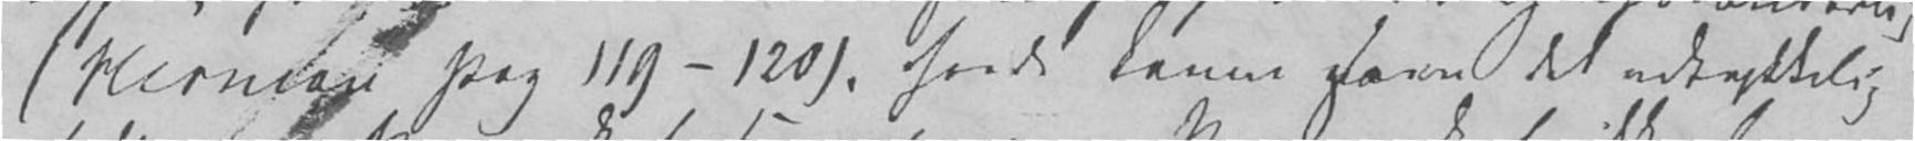(Hernion Pag 119 - 120), fordi denne som det udtrykkelig
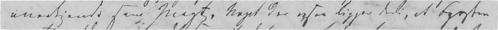anerkjendt som Magt. Noget der ogsaa ligger deri, at Fyrsten
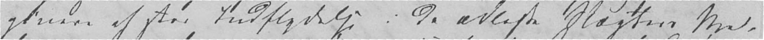givere af stor Indflydelse i de ældste Slægters Me-
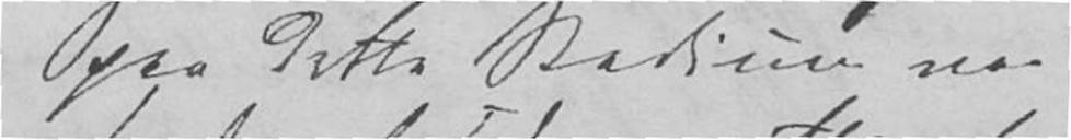paa dette Stadium var
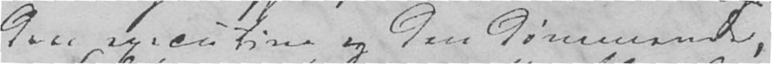den executive og den dømmende,
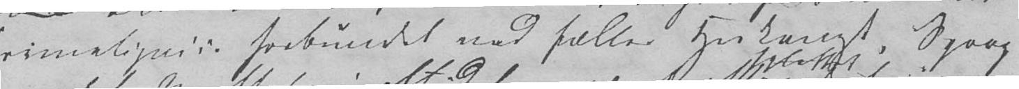rimeligviis forbundet med fælles Herkomst, Sprog
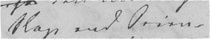Slags end Orien-
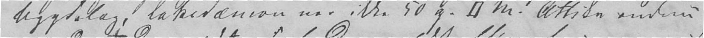Bygdelag, Lakedæmon var ikke 50 K. 8 M. Attika endnu
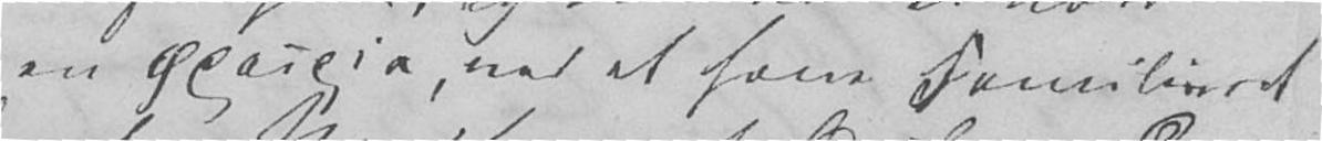en X, ved at have Familieret-
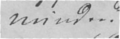mindre.
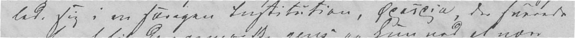lede sig i en særegen Institution, X, der svarede
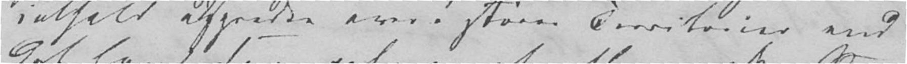ialfald adspredte over større Territorier end
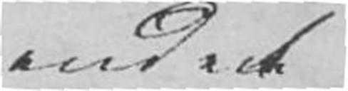andet
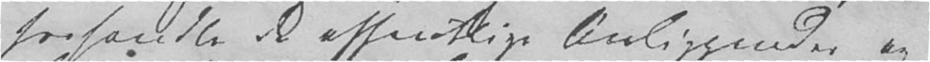forhandle de offentlige Anliggender og
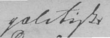politiske
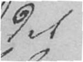det
